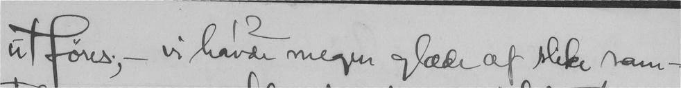utføres,- vi havde megen glæde af slike sam-
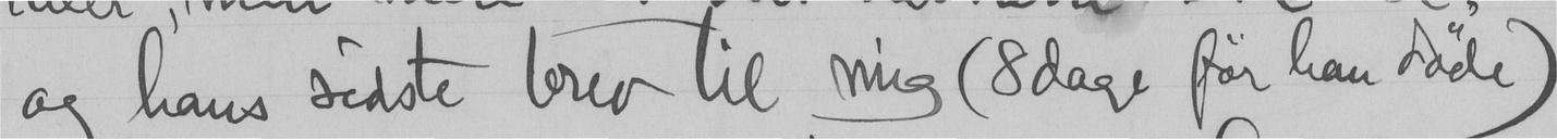og hans sidste brev til mig (8 dage før han døde)
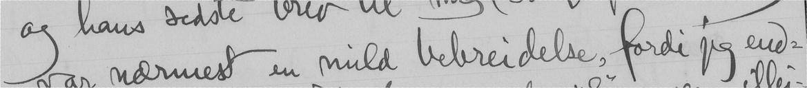var nærmest en mild bebreidelse, fordi jeg end-
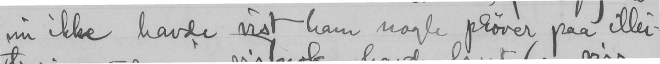nu ikke havde vist ham nogle prøver paa illu-
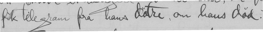fik telegram fra hans døtre, om hans død.
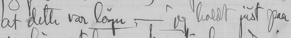at dette var løgn - jeg holdt jast paa
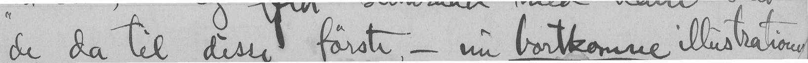de da til disse første - nu bortkomne illustrationer
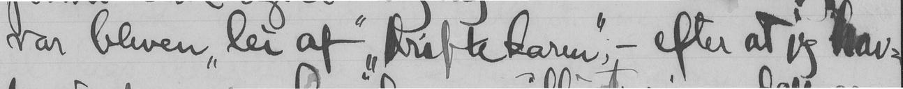var bleven "lei af" "Driftekaren", - efter at jeg hav-
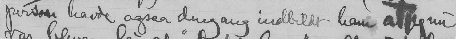person havde ogsaa dengang indbildt ham at jeg nu
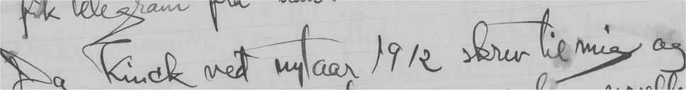Da Kinck ved nytaar 1912 skrev til mig og
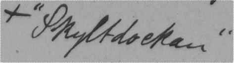x "Skyltdockan"
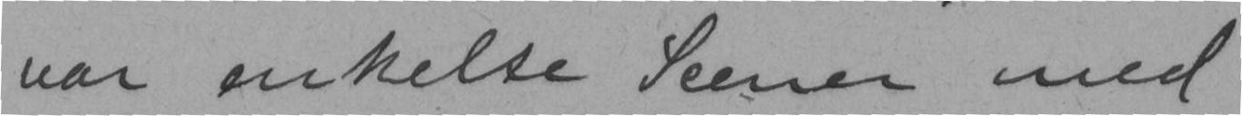var enkelse Scener med
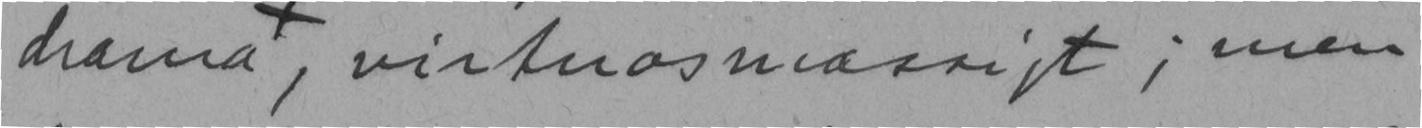drama, virtuosmæssigt; men
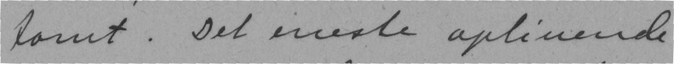tomt. Det eneste oplivende
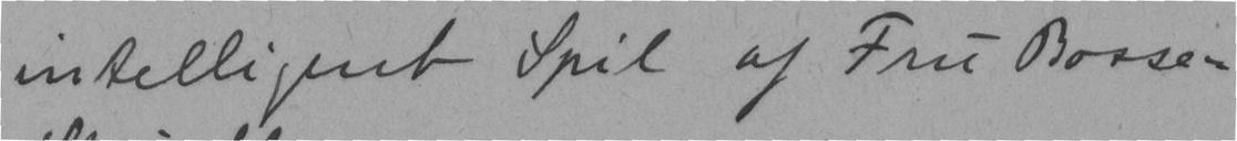intelligent Spil af Fru Bosse-
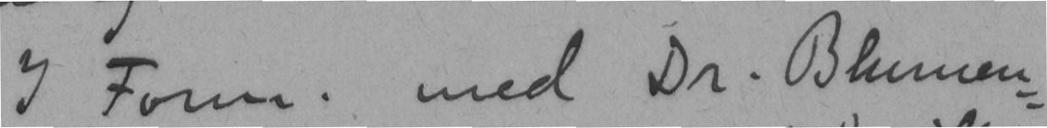I Form. med Dr. Blumen-
